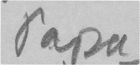Papa
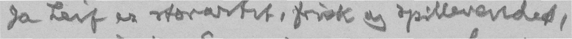Ja Leif er storartet, frisk og spillevendet,
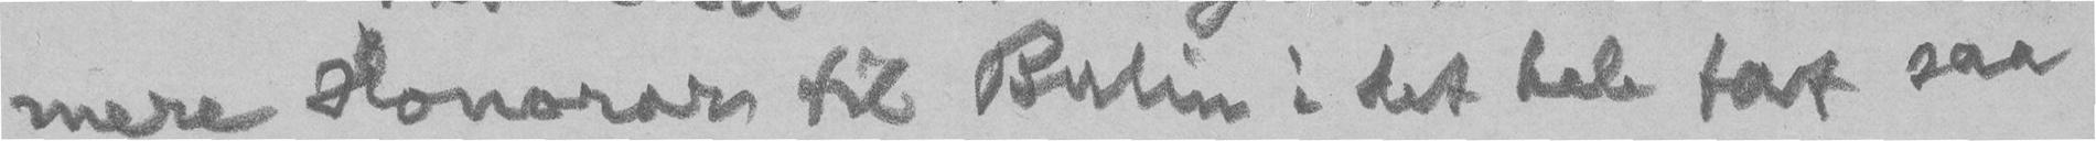mere Honorar til Berlin i det hele tat saa
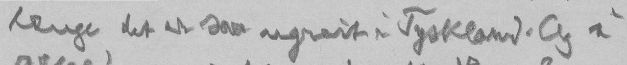længe det er saa ugreit i Tyskland. Og i
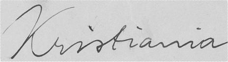Kristiania
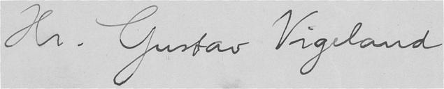Hr. Gustav Vigeland
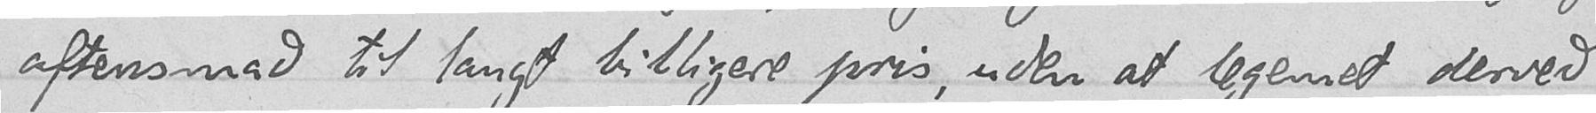aftensmad til langt billigere pris, uden at legemet derved
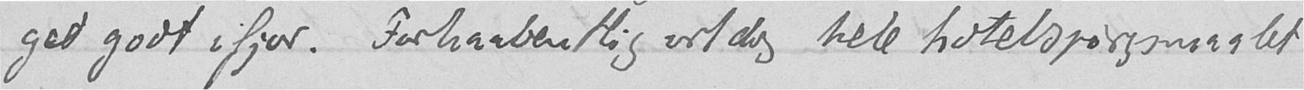get godt ifjor. Forhaabentlig vil dog hele hotelspørgsmaalet
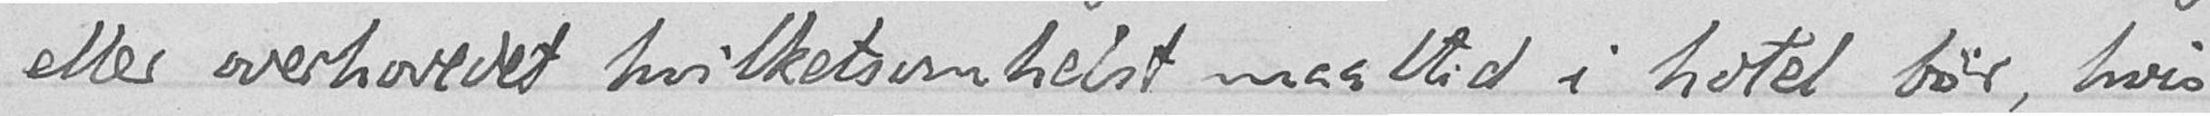eller overhovedet hvilketsomhelst maaltid i hotel bør, hvis
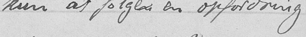kun at følge en opfordring
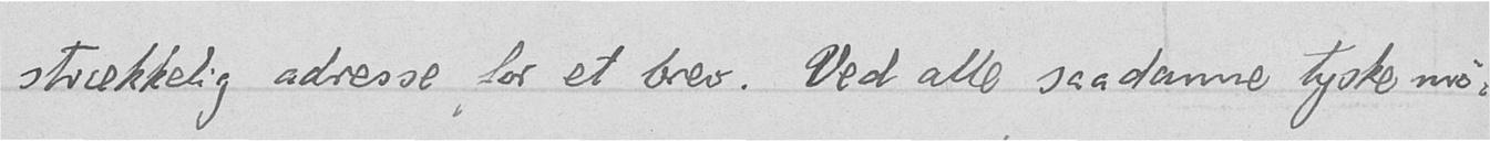strækkelig adresse for et brev. Ved alle saadanne tyske mø-
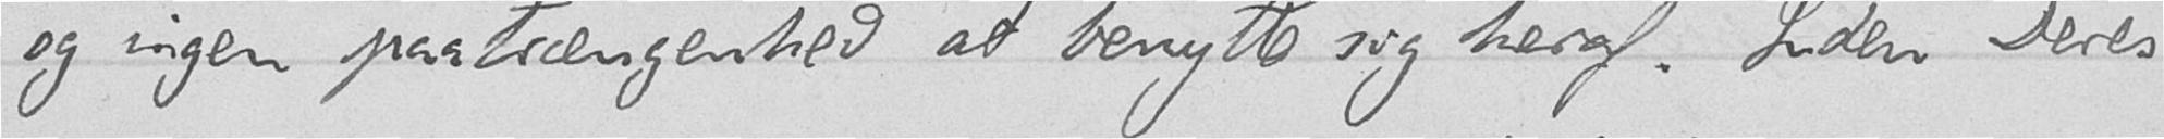og ingen paatrængenhed at benytte sig heraf. Inden Deres
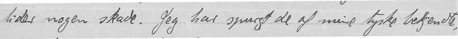lider nogen skade. Jeg har spurgt de af mine tyske bekjendte,
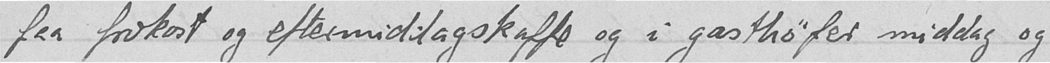faa frokost og eftermiddagskaffe og i gasthöfer middag og
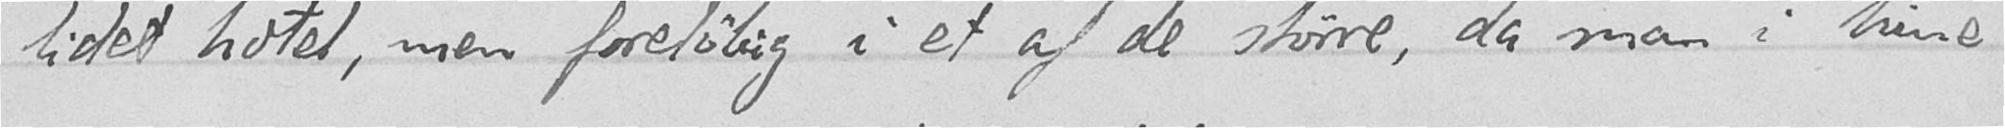lidet hotel, men foreløbig i et af de store, da man i hine
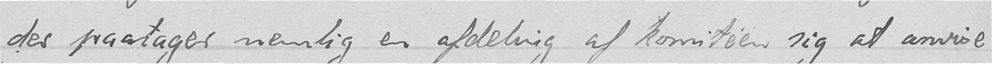der paatager nemlig en afdeling af komitéen sig at anvise
